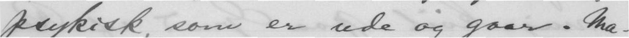psykisk, som er ude og gaar. Ma-
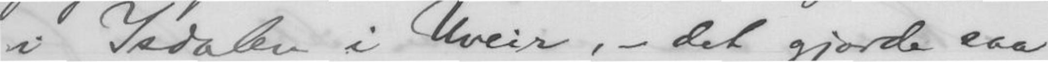i Isdalen i Uveir, - det gjorde saa
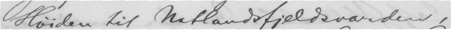Høiden til Natlandsfjeldsvarden,
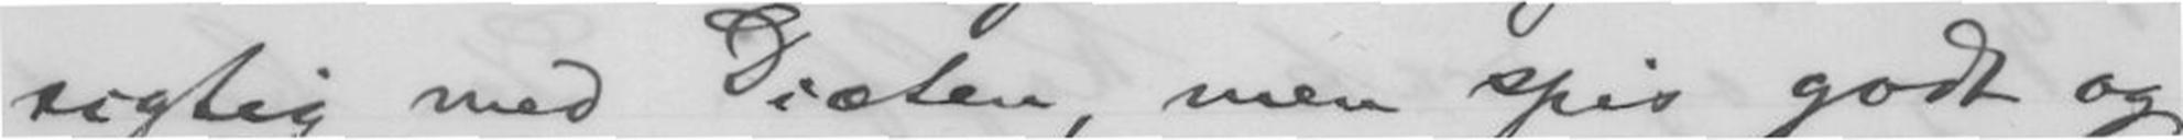sigtig med Diæten, men spis godt og
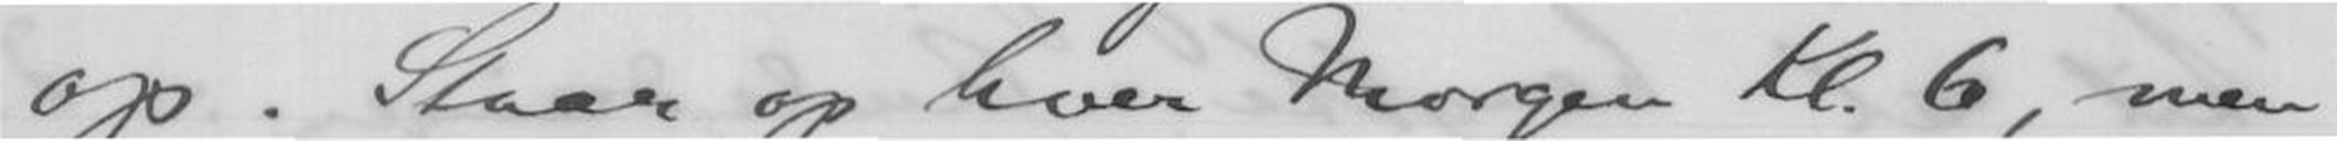op. Staar op hver Morgen Kl 6, men
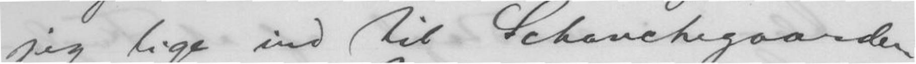jeg lige ind til Schanchegaarden
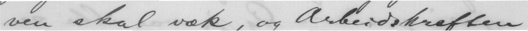ven skal væk, og Arbeidskraften
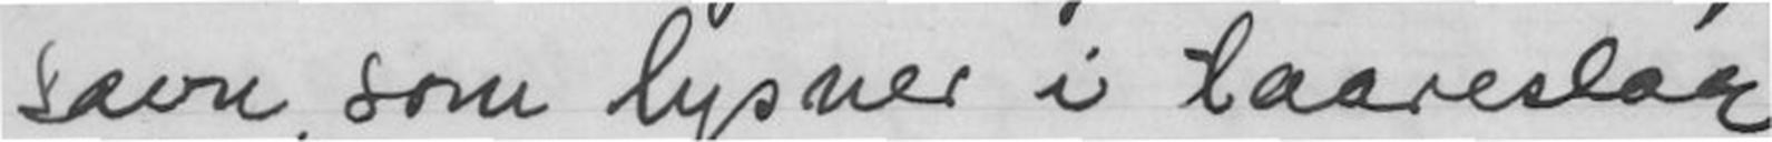savn, som lysner i taareslør
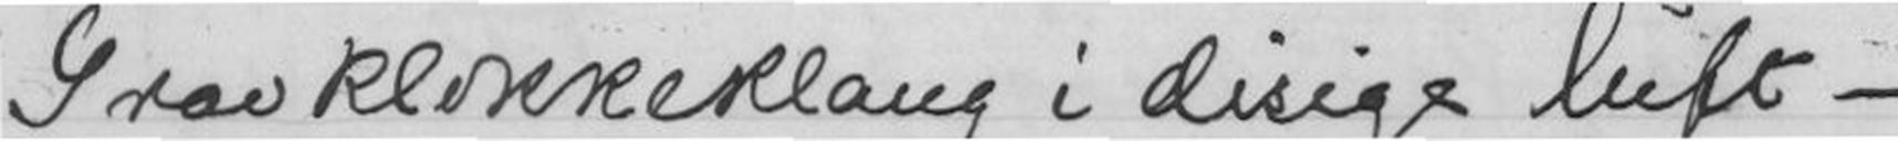Gravklokkeklang i disige luft -
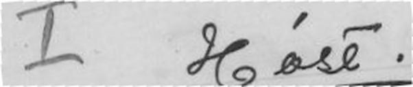I Høst.
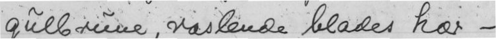gulbrune, raslende blades hær -
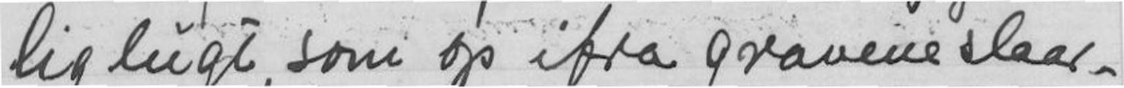liglugt, som op ifra gravene slaar -
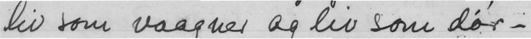liv som vaagner og liv som dør -
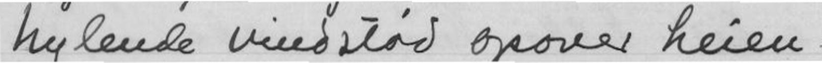hylende vindstød opover heien
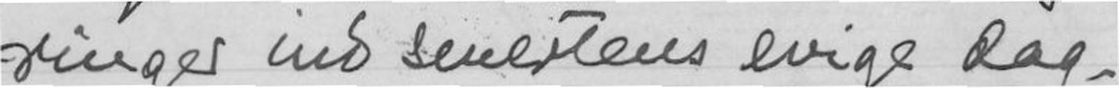ringer ind senerlens evige dag -
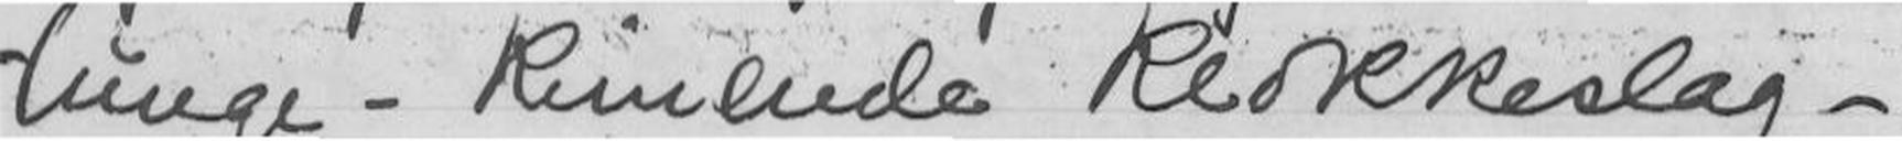tunge - Kimende klokkeslag -
